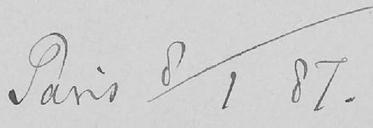Paris 8/1 87.
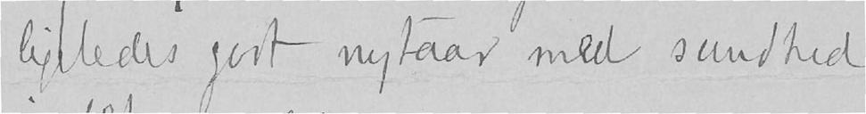ligeledes godt nytaar med sundhed
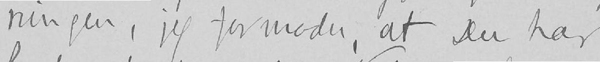ningen, jeg formoder, at Du har
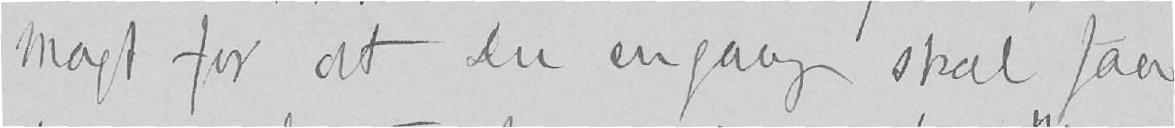magt for at Du engang skal faa
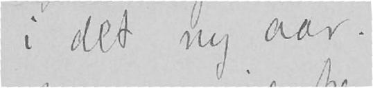i det ny aar.
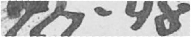9/7-48
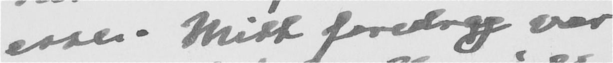esse. Mitt foredrag var
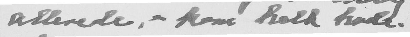allerede, - kom helt hode-
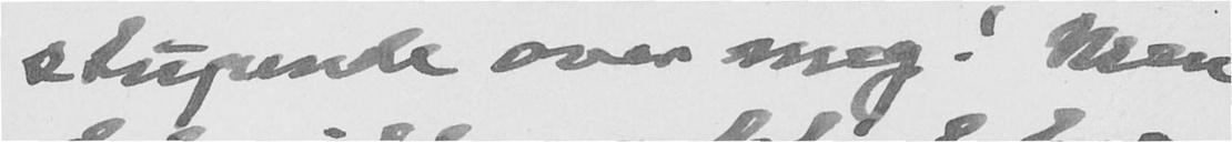stupende over meg! Men
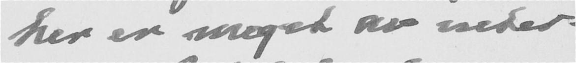her er meget av inter-
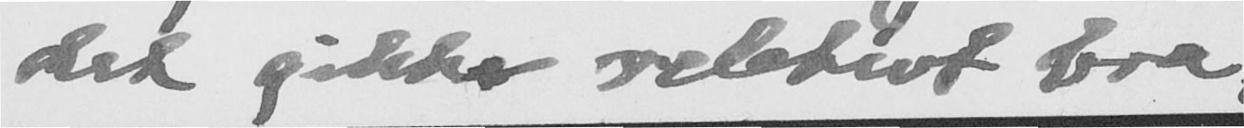det gikk relativt bra.
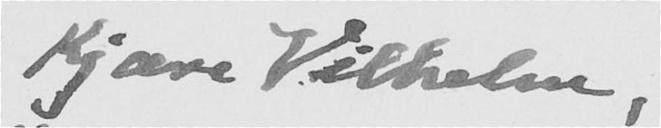Kjære Vilhelm,
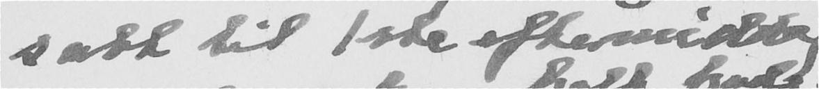satt til 1ste eftermiddag
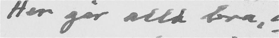Her går allt bra, og
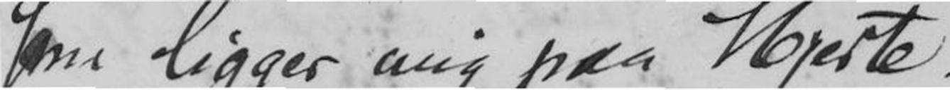som ligger mig paa Hjerte.
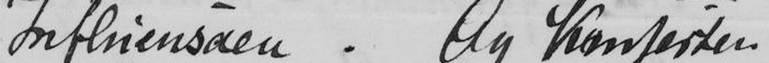Influensaen. Og Konserten
